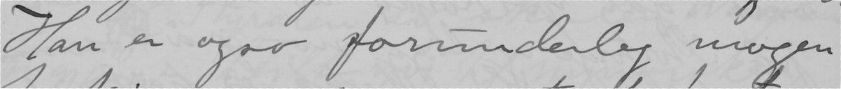Han er også forunderleg mogen
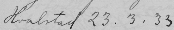Hvalstad 23.3.33
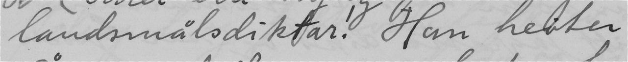landsmålsdiktar. Han heiter
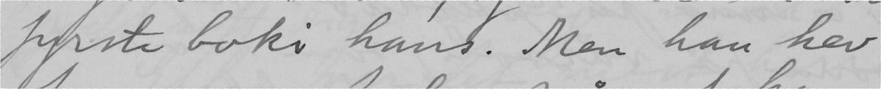förste boki hans. Men han hev
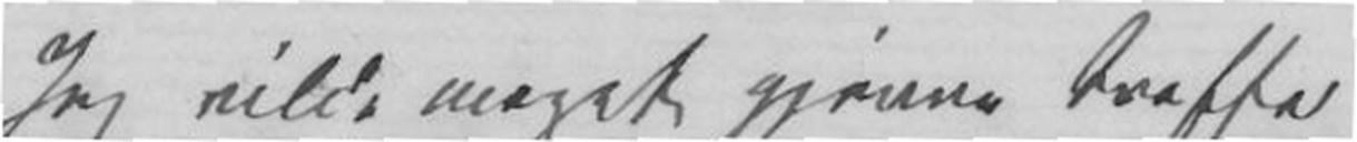Jeg vilde meget gjerne treffe
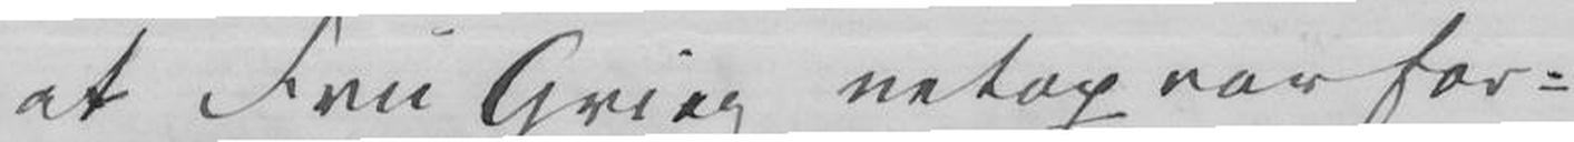at Fru Grieg netop var for-
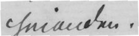hinanden.
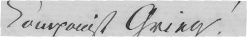Komponist Grieg!
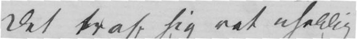Det traf sig ret uheldig
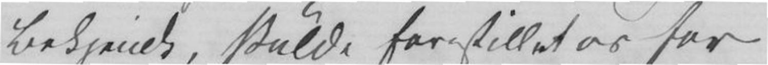Bekjendt, skulde forestillet os for
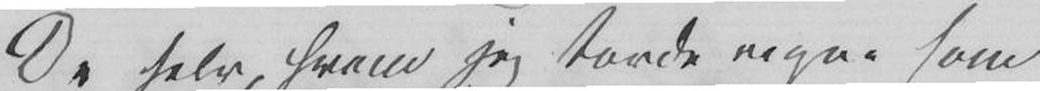De selv, hvem jeg torde regne som
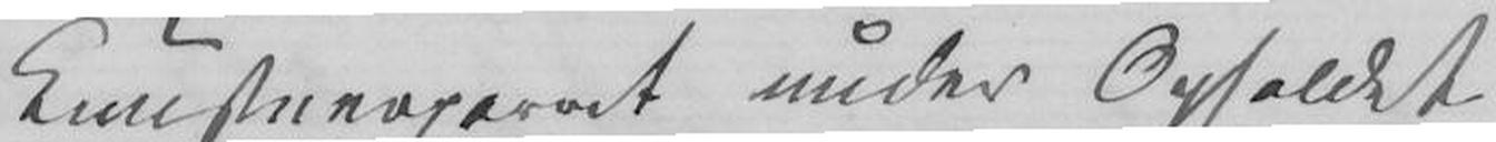Kunstnerparet under Opholdet
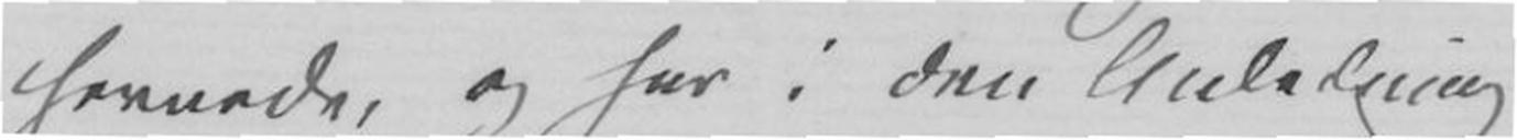hernede, og har i den Anledning
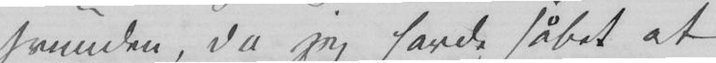svunden, da jeg havde håbet at
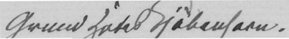Grand Hotel Kjøbenhavn.
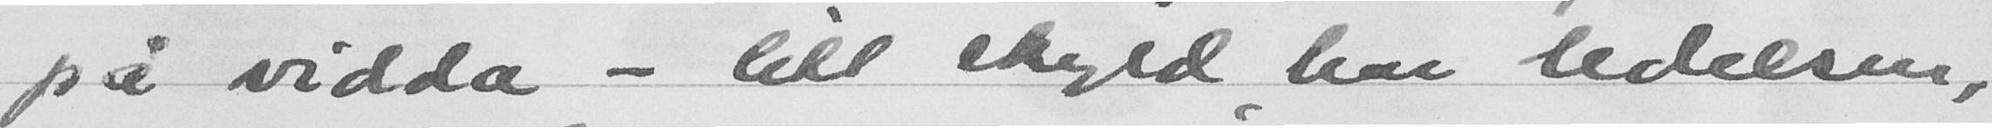på vidda - litt skyld har ledelsen,
